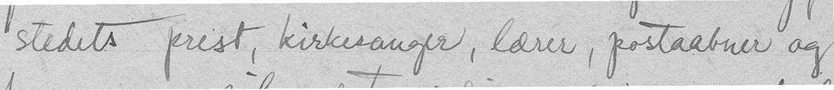stedets prest, kirkesanger, lærer, postaabner" og
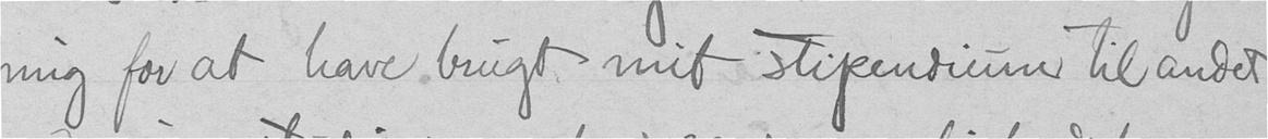mig for at have brugt- mit stipendium til andet
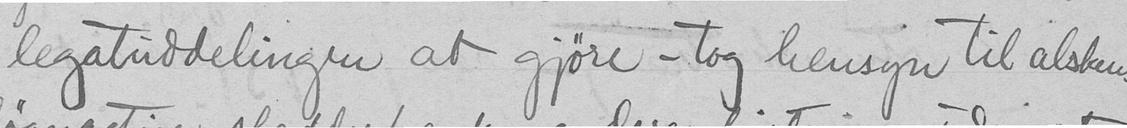legatuddelingen at gjøre - tog hensyn til alskens
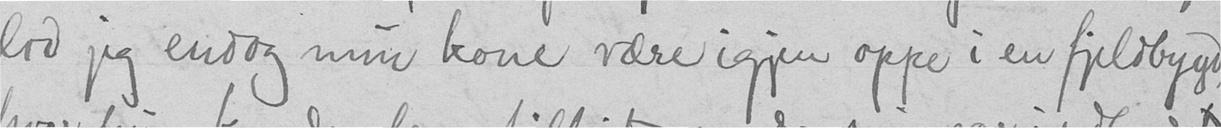lod jeg endog min kone være igjen oppe i en fjeldbygd,
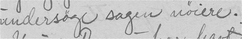udersøge sagen nøiere.
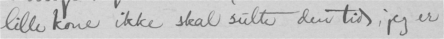lille kone ikke skal sulte den tid, jeg er
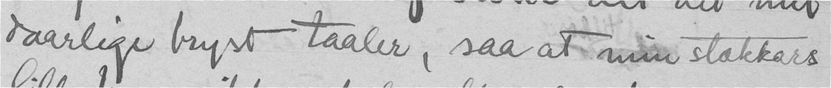daarlige bryst taaler, saa at min stakkars
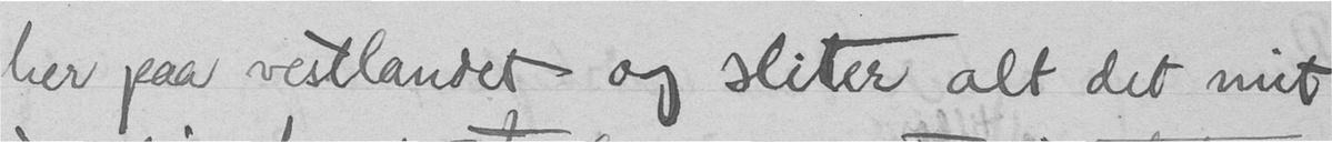her paa vestlandet og sliter alt det mit
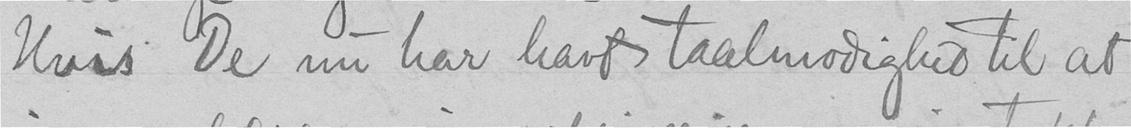Hvis De nu har havt taalmodighed til at
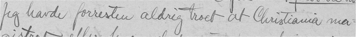Jeg havde forresten aldrig troet at Christiania ma-
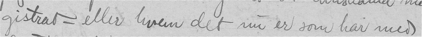gistrat- eller hvem det nu er som har med
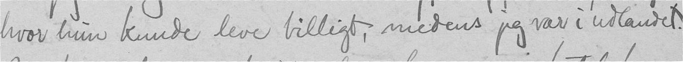hvor hun kunde leve billigt medens jeg var i udlandet.
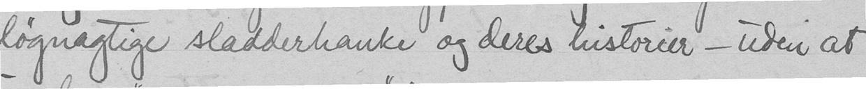løgnagtige sladderhanke og deres historier - uden at
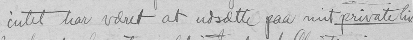intet har været at udsætte paa mit private liv.
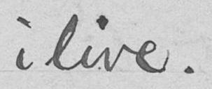i live.
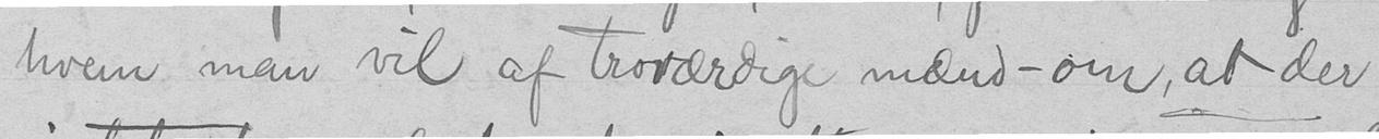hvem man vil af troværdige mænd - om, at der
